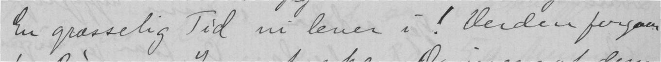En græsselig Tid vi lever i! Verden forgaar
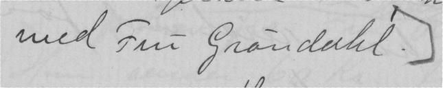med Fru Grøndahl.
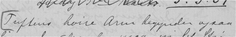Tuftens høire arm begynder ogsaa
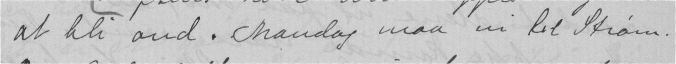at bli ond. Mandag maa vi til Strøm.
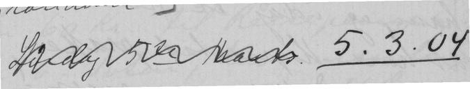. 5.3.04
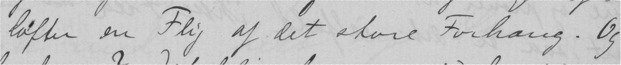løfter en Flig af det store Forhæng. Og
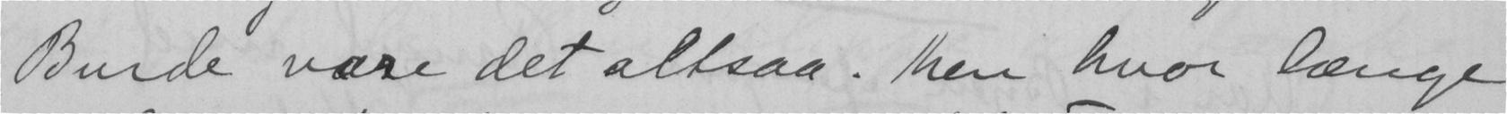Burde være det altsaa. Men hvor længe
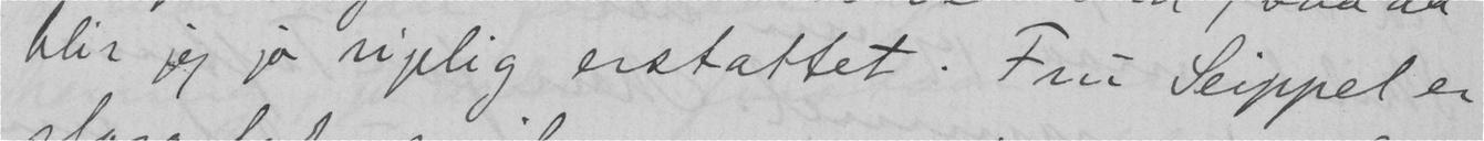blir jeg jo rigelig erstattet. Fru Seippel er
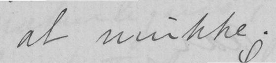at mukke.
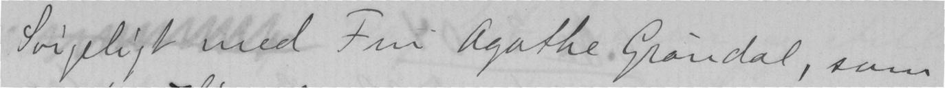Sørgeligt med Fru Agathe Grøndal, som
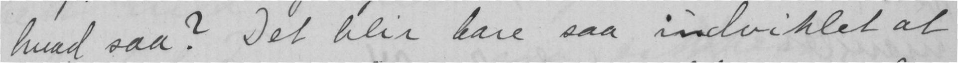hvad saa? Det blir bare saa indviklet at
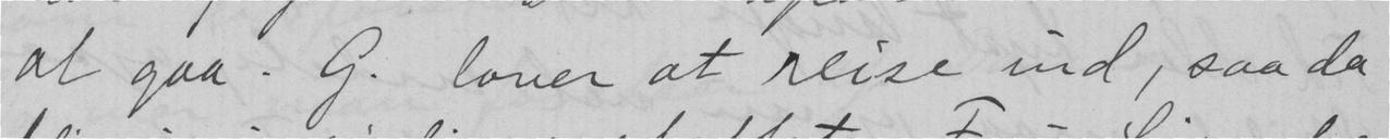at gaa. G. lover at reise ind, saa da
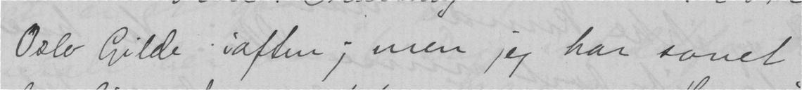Oslo Gilde iaften; men jeg har sovet
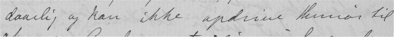daarlig og kan ikke opdrive Humør til
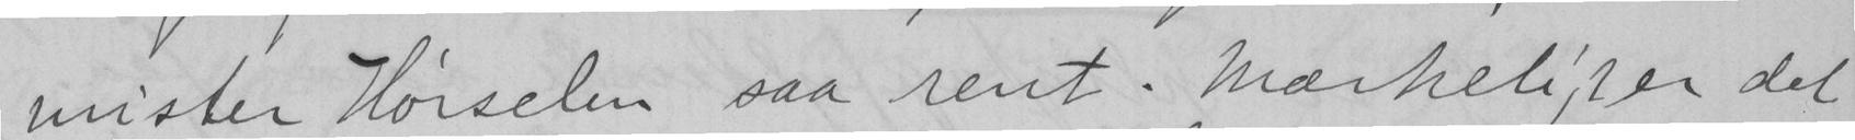mister Hørselen saa rent. Mærkeligt er det
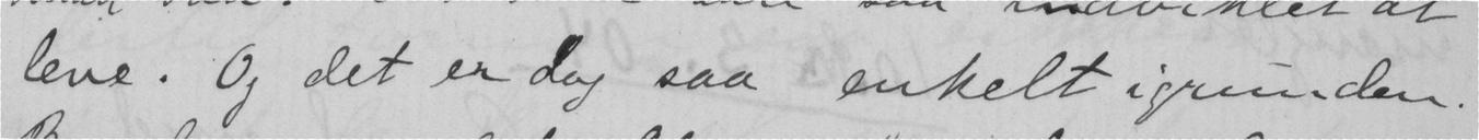leve. Og det er dog saa enkelt igrunden.
